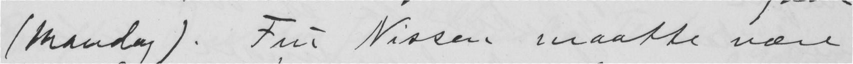(Mandag). Fru Nissen maatte være
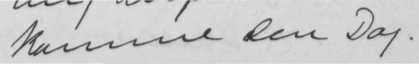komme den Dag.
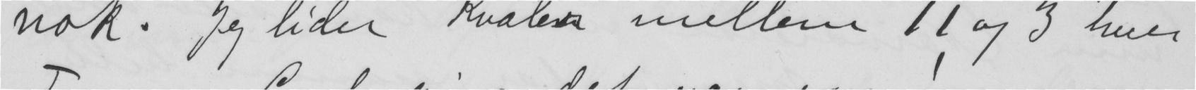nok. Jeg lider Kvaler mellem 11 og 3 hver
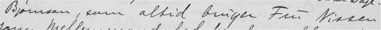Bjørnson, som altid bruger Fru Nissen
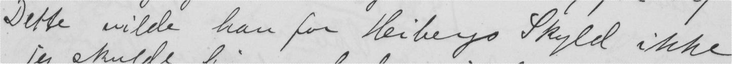Dette vilde han for Heibergs Skyld ikke
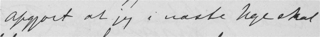Afgjort at jeg i næste Uge skal
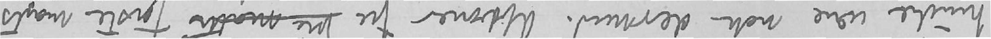kunde være nok dermed. Abboner fra maths første marts
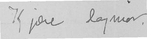Kjære Dagmar.
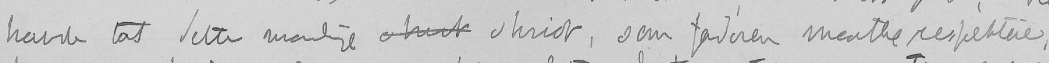havde tat dette mandige skriit skridt, som faderen, maatte respektere,
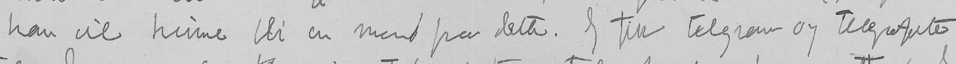han vil kunne bli en mand paa dette. Jeg fik telegram og telegraferte
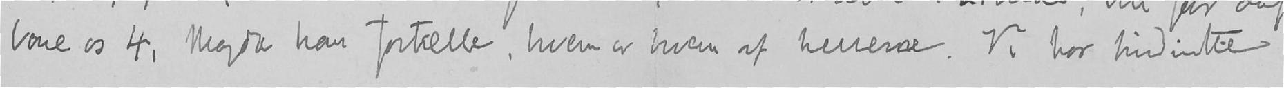bare os 4, Magda kan fortælle, hvem er hvem af herrerne. Vi har hidentil
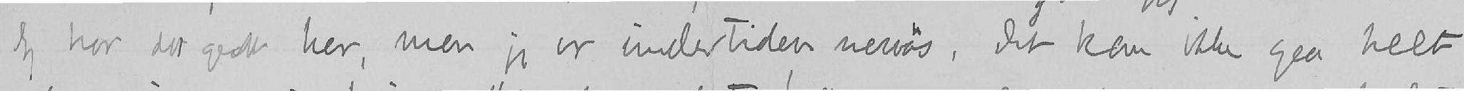Jeg har det godt her, men jeg er undertiden nervøs, det kan ikke gaa helt
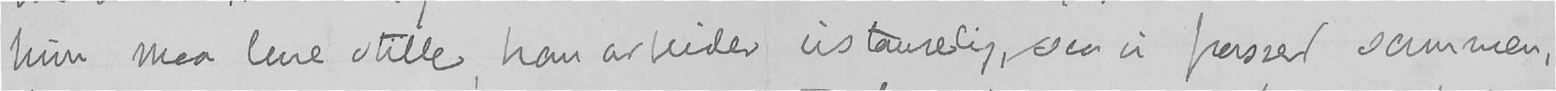hun maa leve stille, han arbeider ustanselig saa vi passer sammen.
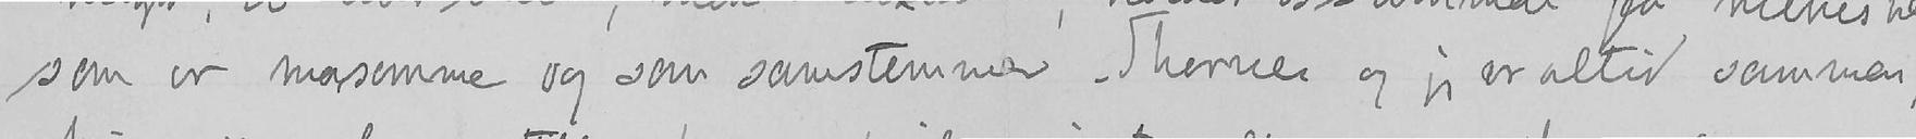som er morsomme og som samstemmer. Thornes og jeg er altid sammen,
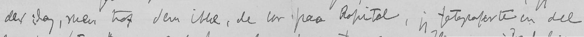der idag, men traf dem ikke, de bor paa Kapitol, jeg fotograferte en del
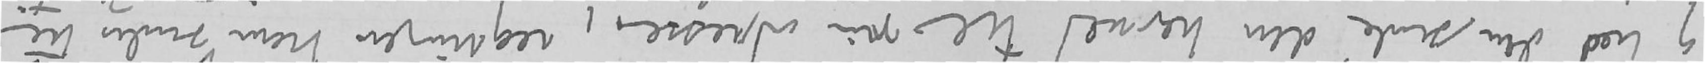og bed dem sende den herned til min adresse, regningen kan sendes til
